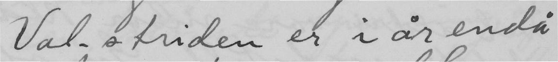Val-striden er i år endå
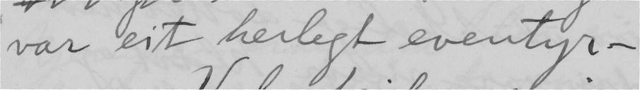var eit herlegt eventyr –
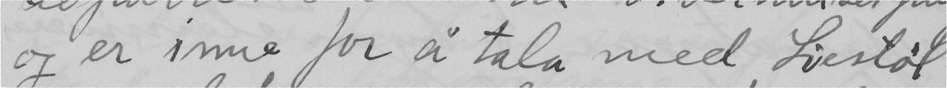og er inne for å tala med Lierstøl
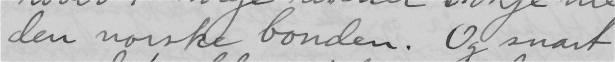den norske bonden. Og snart
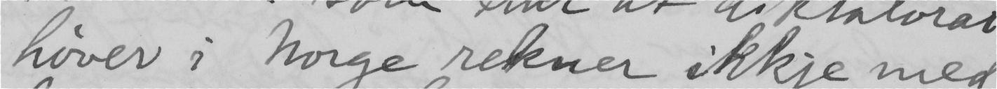höver i Norge rekner ikkje med
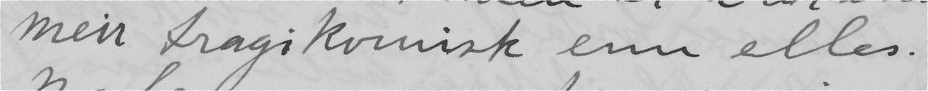Meir tragikomisk enn elles.
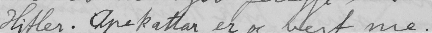Hitler. Apekattar er og vert me.
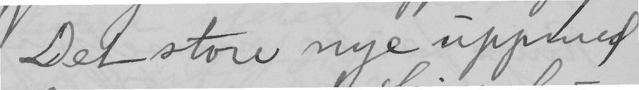Det store nye uppmed
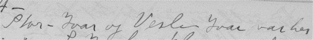Stor-Ivar og Vesle-Ivar var her
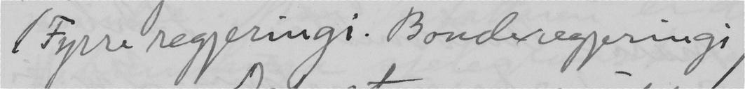(Fyrre regjeringi. Bonderegjeringi)
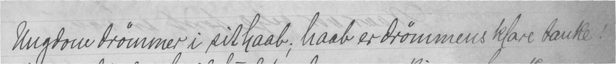Ungdom drømmer i sit haab; haab er drømmens klare tanke!
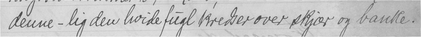denne - lig den hvide fugl kredser over skjær og banke.
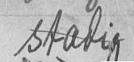stadig
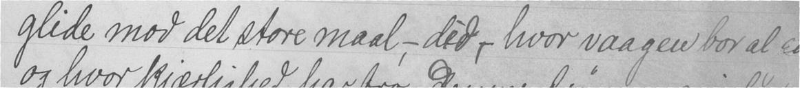glide mod det store maal, - did, - hvor vaagen bor al
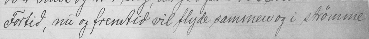Fortid, nu og fremtid vil flyde sammen og i strømme
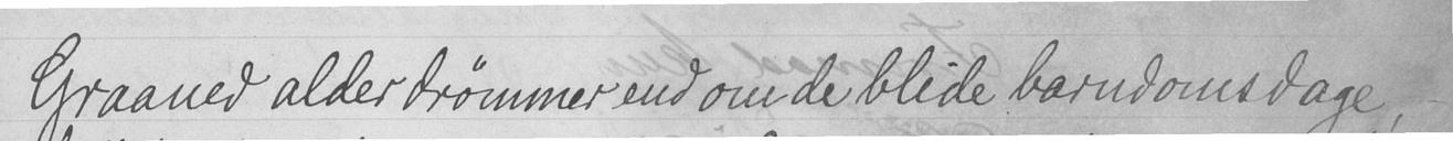Graaned alder drømmer end om de blide barndomsdage, -
